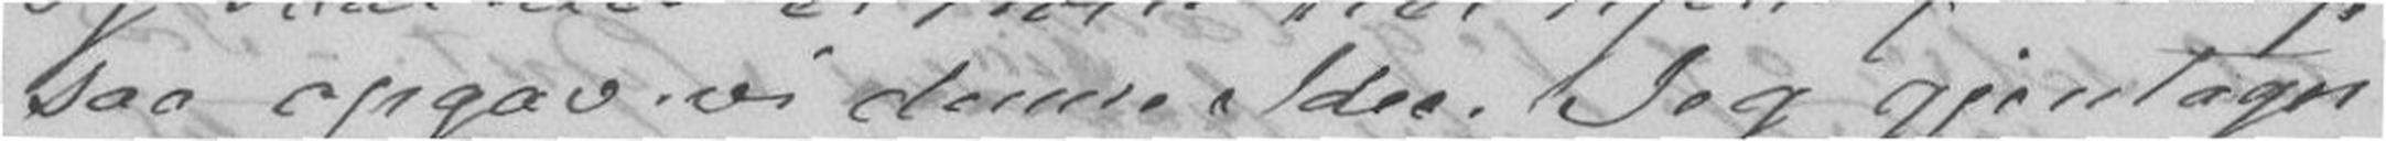saa opgav vi denne Idee. Jeg gjentager
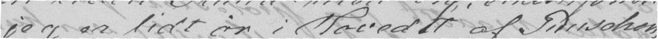jeg er lidt ør i Hovedet af Punchen,
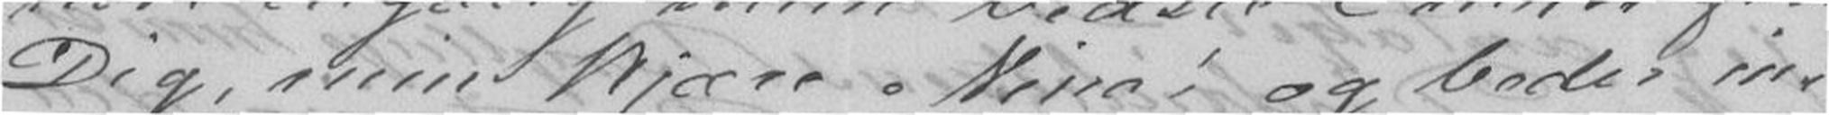Dig, min kjære Nina! og beder in-
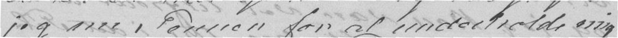jeg nu Pennen for at underholde mig
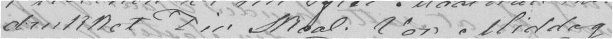drukket Din Skaal. Vor Middag
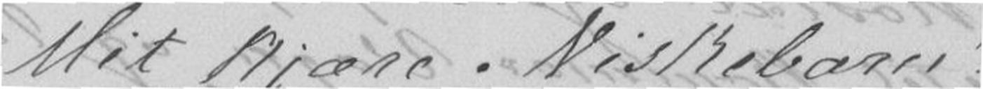Mit kjære Niskebarn!
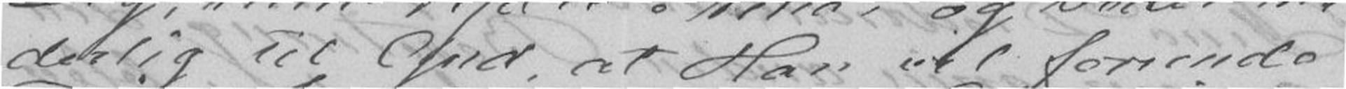derlig til Gud at Han vil forunde
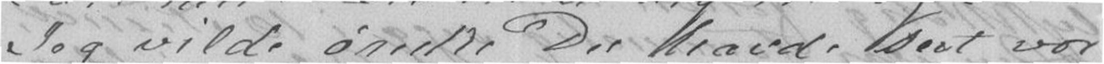Jeg vilde ønske Du havde seet vor
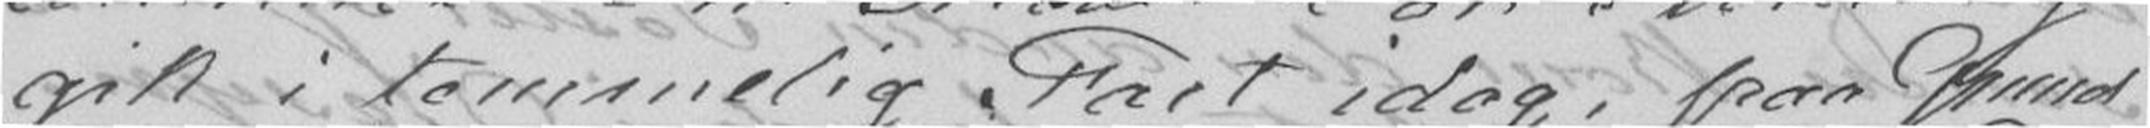gik i temmelig Fart idag, paa Grund
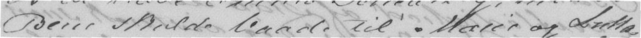Bene skulde baade til Marie og Lulla
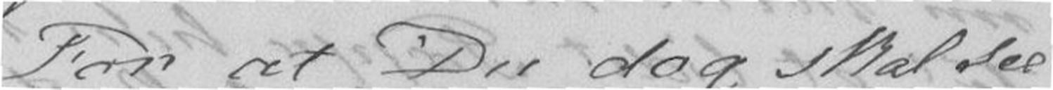For at Du dog skal see
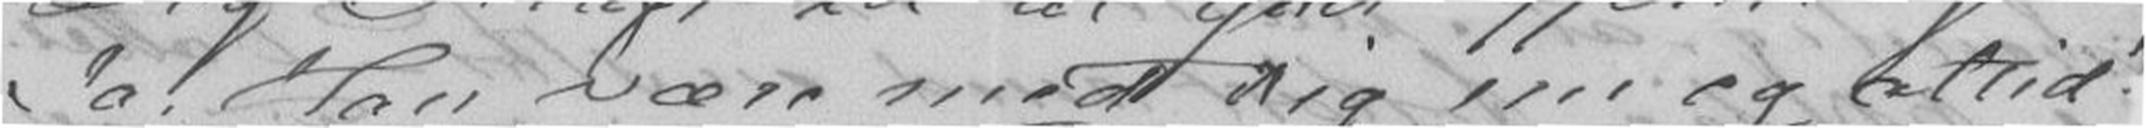Ja, Han være med Dig nu og altid.
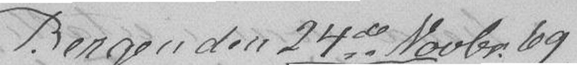Bergen den 24de novbr. 69
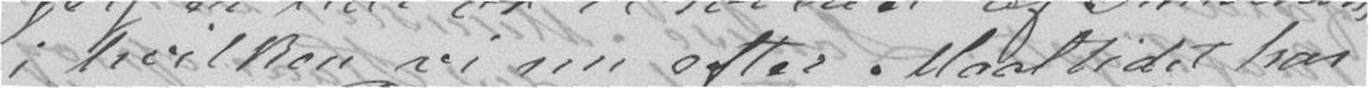i hvilken vi nu efter Maaltidet har
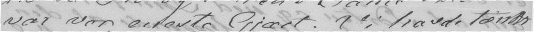var vor eneste Gjæst. Vi havde tænkt
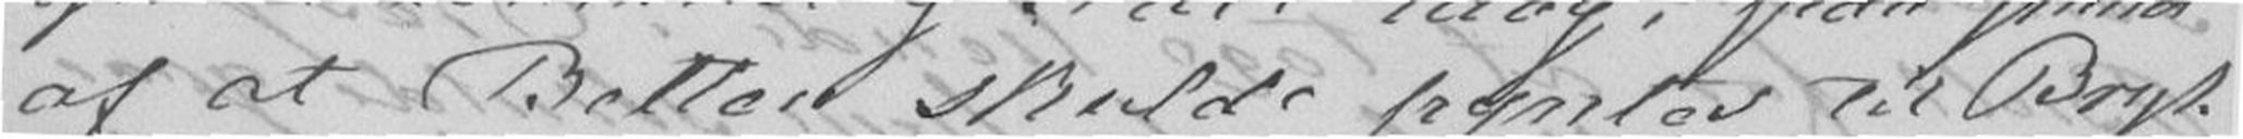af at Betten skulde pyntes til Bryl-
